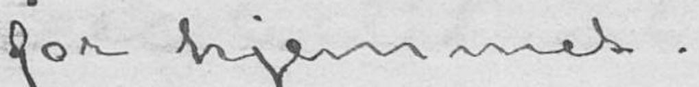for hjemmet.
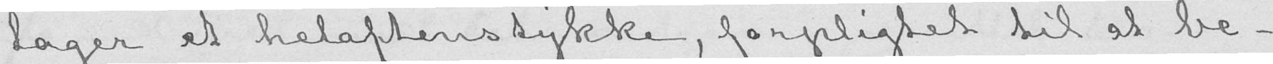tager et helaftenstykke, forpligtet til at be-
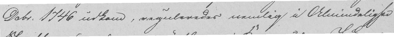Dcbr. 1746 udkom, reguleredes nemlig i Almindelighed
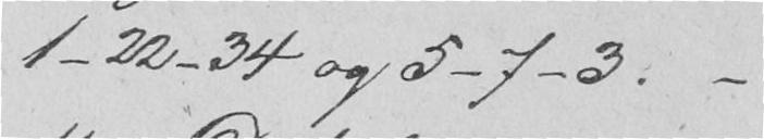1-22-34 og 5-7-3. -
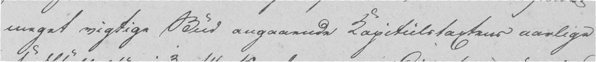meget vigtige Bud angaaende Kapitulstaxtens aarlige
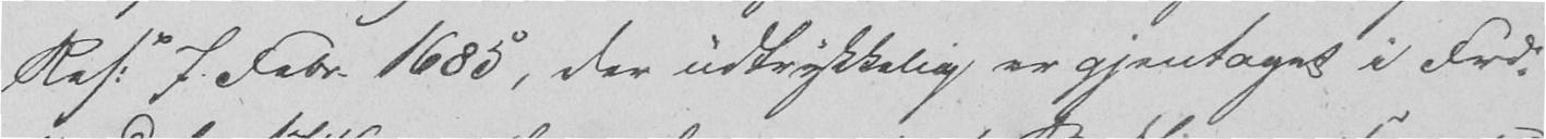Res. 7. Febr. 1685, der udtrykkelig er gjentaget i Frdn
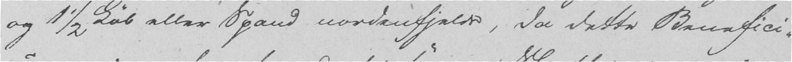og 1 1/2 Løb eller Spand nordenfjelds, da dette Benefici-
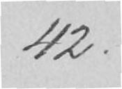42.
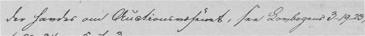der havdes om Auctionsvæsenet, see Lovbogens 3-19-23,
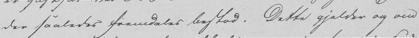der saaledes fremdeles bestod. Dette gjelder og om
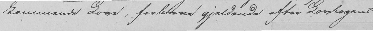kommende Love, forblive gjeldende efter Lovbogens
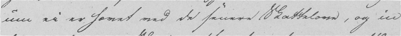um ei er hævet ved de senere Skattelove, og in
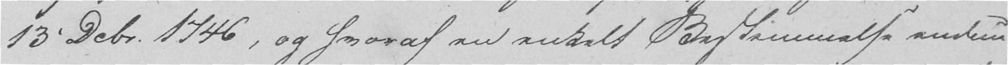13. Dcbr. 1746, og hvoraf en enkelt Bestemmelse endnu
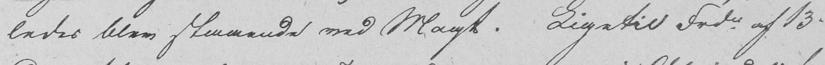ledes blev staaende ved Magt. Ligetil Frdn af 13.
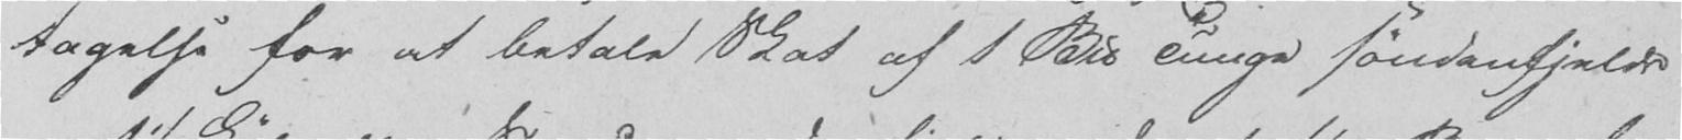tagelse for at betale Skat af 1 Bes Tunge søndenfjelds
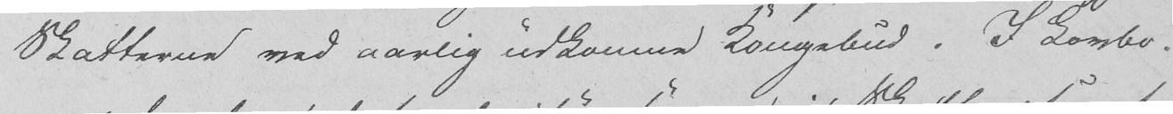Skatterne ved aarlig udkomne Kongebud. I Lovbo-
